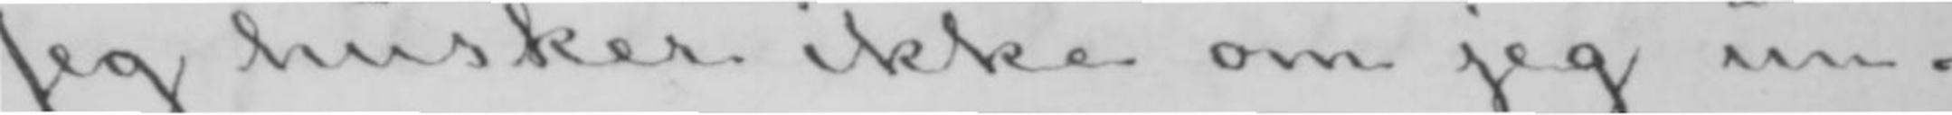Jeg husker ikke om jeg un-
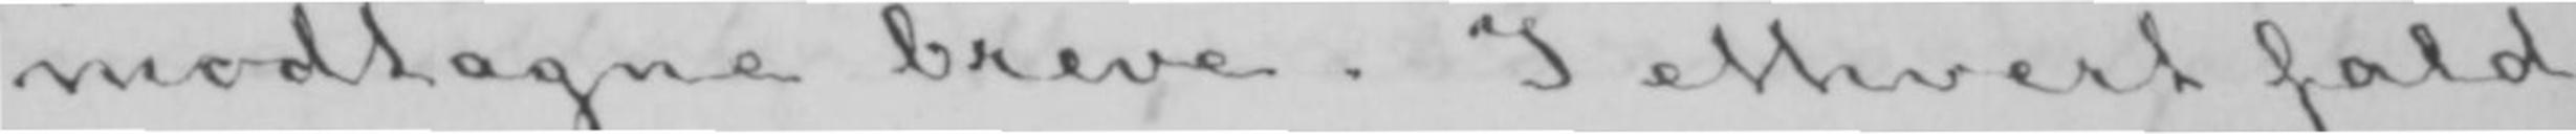modtagne breve. I ethvert fald
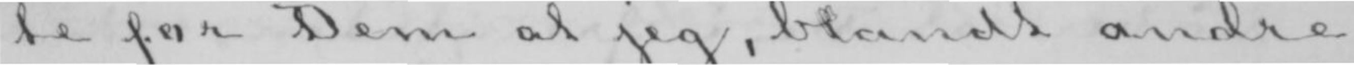te for Dem at jeg, blandt andre
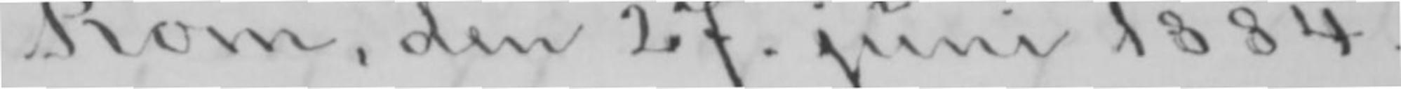Rom, den 27. juni 1884.
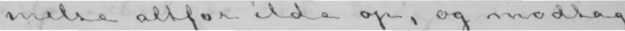melse altfor ilde op, og modtag
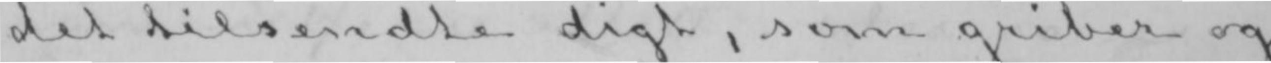det tilsendte digt, som griber og
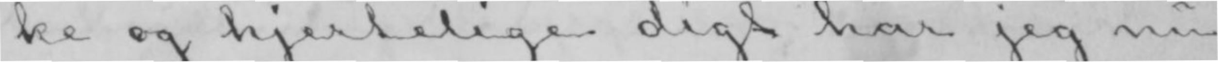ke og hjertelige digt har jeg nu
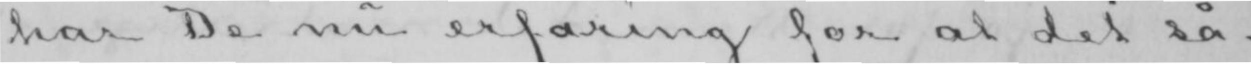har De nu erfaring for at det så-
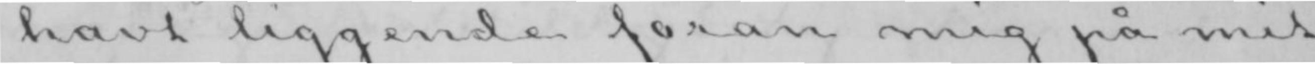havt liggende foran mig på mit
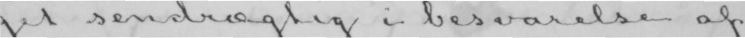get sendrægtig i besvarelse af
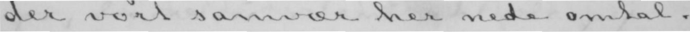der vort samvær her nede omtal-
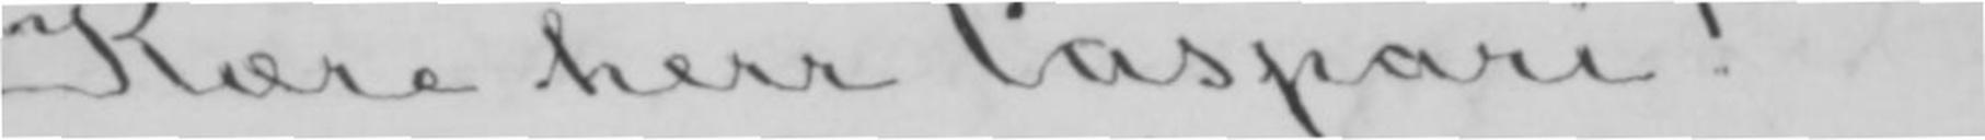Kære herr Caspari!
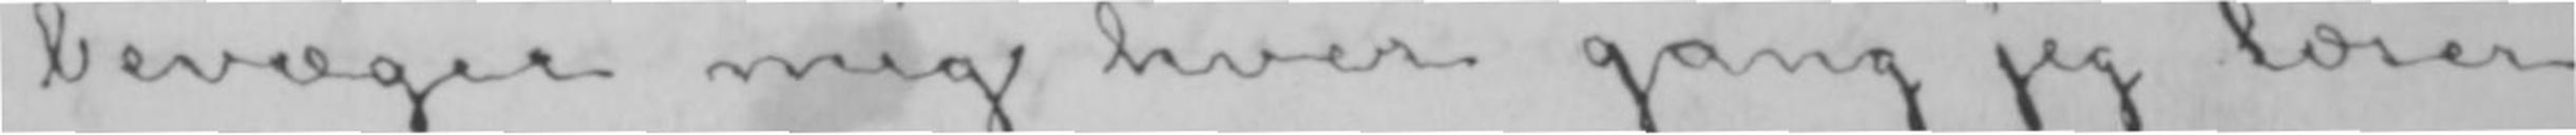bevæger mig hver gang jeg læser
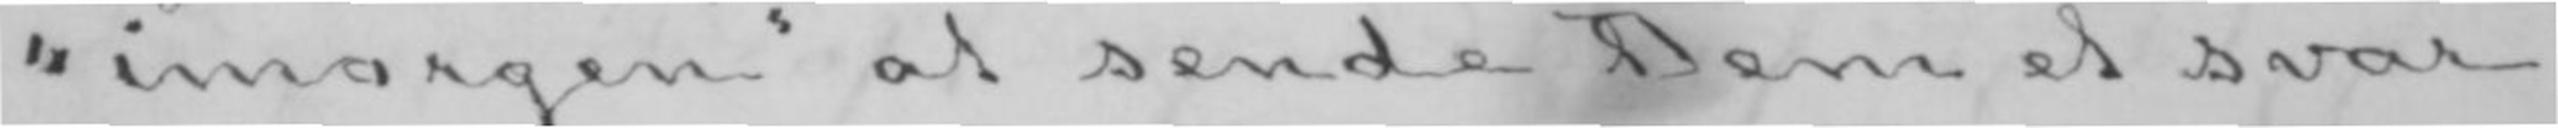«imorgen» at sende Dem et svar.
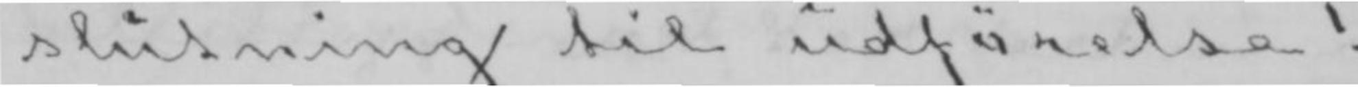slutning til udførelse!
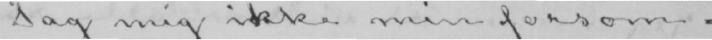Tag mig ikke min forsøm-
